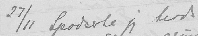27/11 Spadserte jeg trods
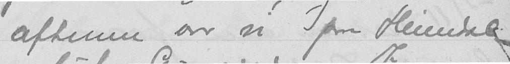aftenen var vi paa Heimdal
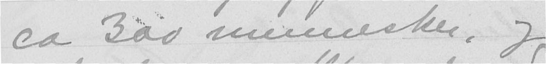ca 300 mennesker, og
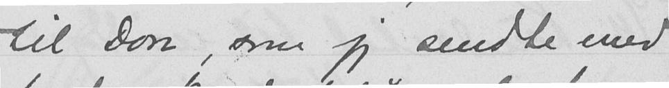til Don, som jeg sendte med
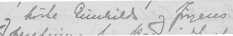og hørte Gunhilds og Jørgens
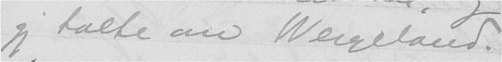jeg talte om Wergeland.
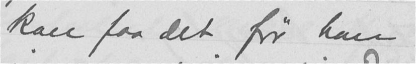kan faa det før han
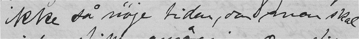ikke så nøje tiden, da man skal
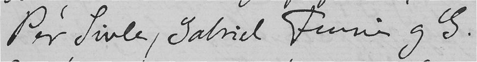Per Sivle, Gabriel Finne og G.
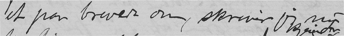et par brever om, skriver jeg nu
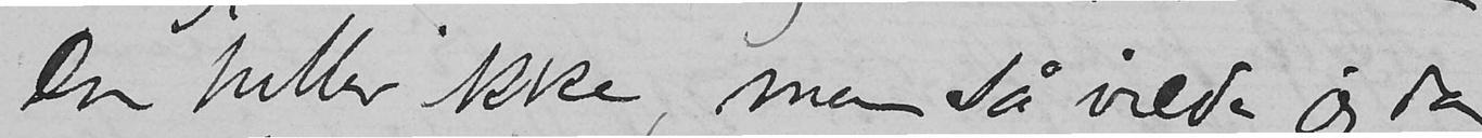en heller ikke, men så vilde jeg da
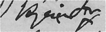kjender
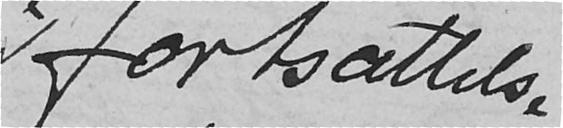fortsættelse
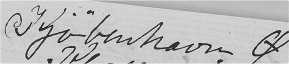Kjøbenhavn Ø
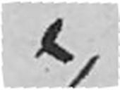i
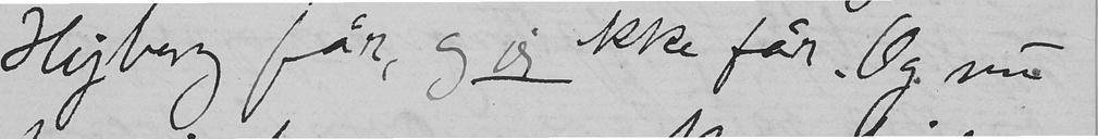Hejberg får, og vi ikke får. Og nu
